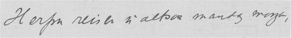Herfra reiser vi altsaa mandag morgen,
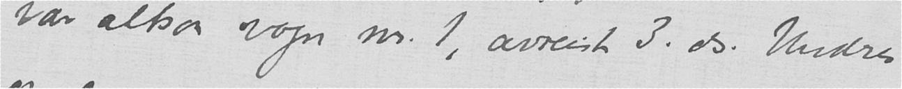var altsaa vogn nr. 1, avreist 3. ds. Undres
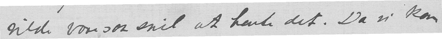vilde være saa snil at hente det. Da vi kom
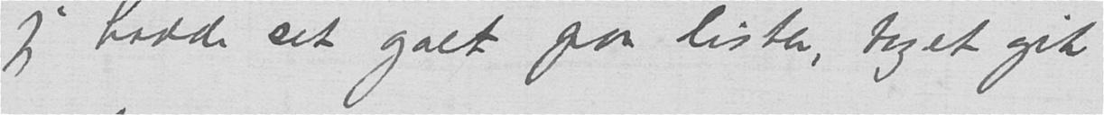jeg hadde set galt paa listen, toget gik
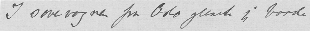I sovevognen fra Oslo glemte jeg baade
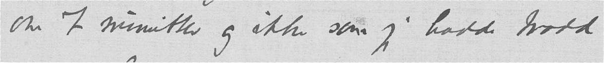om 7 minutter og ikke som jeg hadde trodd
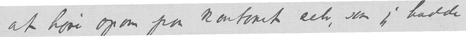at høre opom paa kontoret selv, som jeg hadde
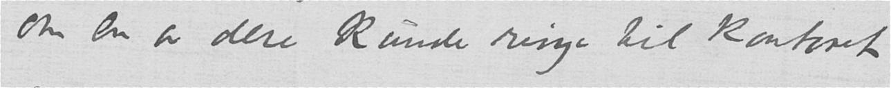om en av dere kunde ringe til kontoret
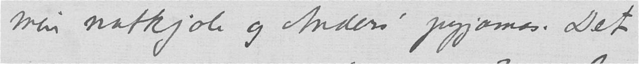min natkjole og Anders’ pyjamas. Det
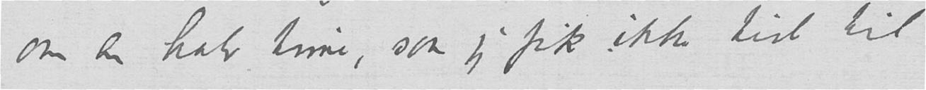om en halv time, saa jeg fik ikke tid til
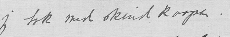jeg tok med skindkaapen.
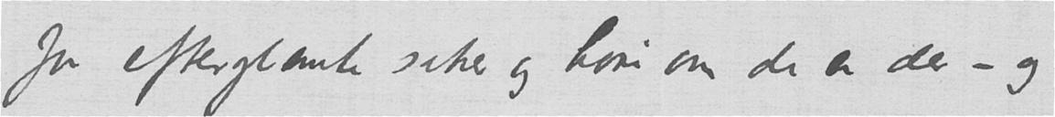for efterglæmte saker og høre om de er der – og
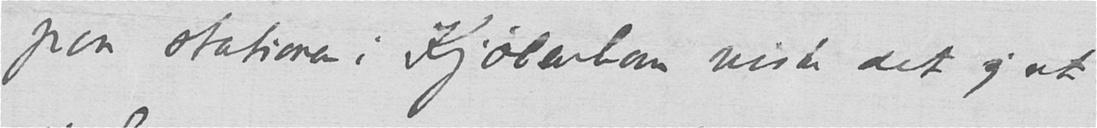paa stationen i Kjøbenhavn viste det sig at
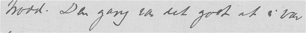trodd. Den gang var det godt at vi var
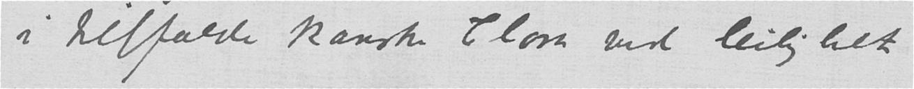i tllfælde kanske Clara ved leilighet
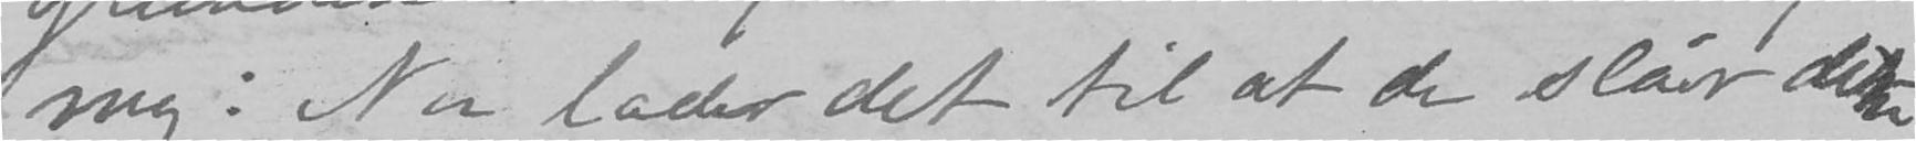mig: Nu lader det til at de slår dem
